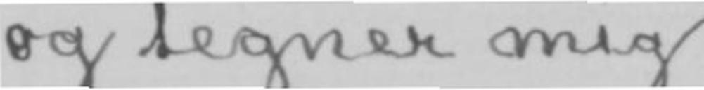og tegner mig
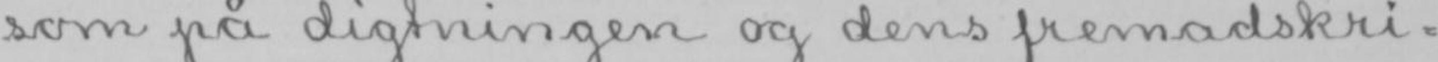som på digtningen og dens fremadskri-
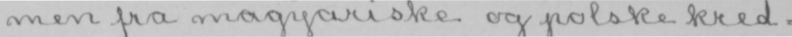men fra magyariske og polske kred-
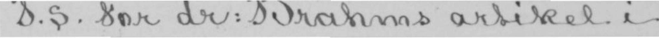P. S. For dr: Brahms artikel i
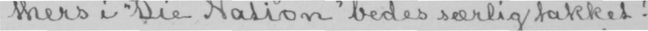-thers i «Die Nation» bedes særlig takket!
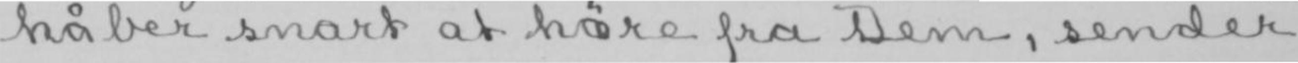håber snart at høre fra Dem, sender
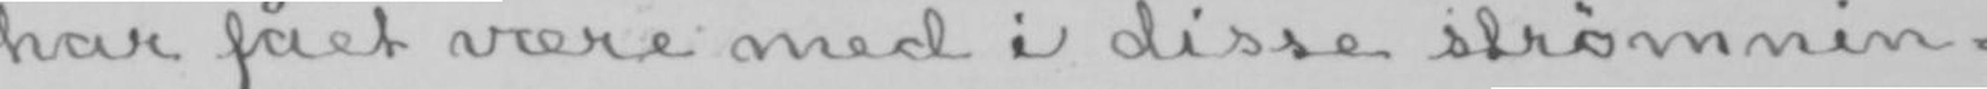har fået være med i disse strømnin-
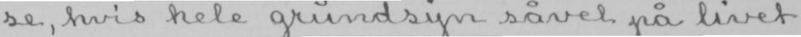se, hvis hele grundsyn såvel på livet
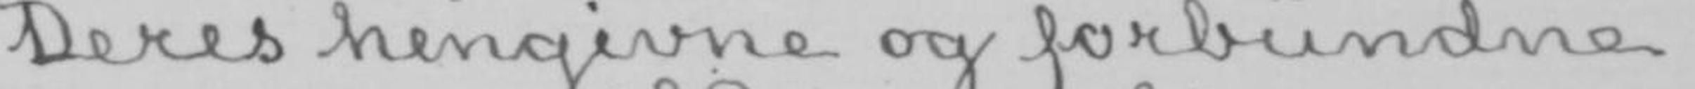Deres hengivne og forbundne
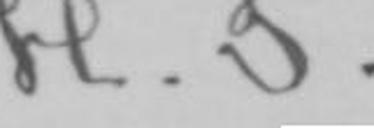H. I.
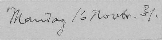Mandag 16 Novbr. 31.
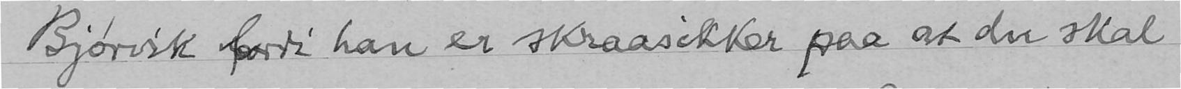Bjørvik fordi han er skraasikker paa at du skal
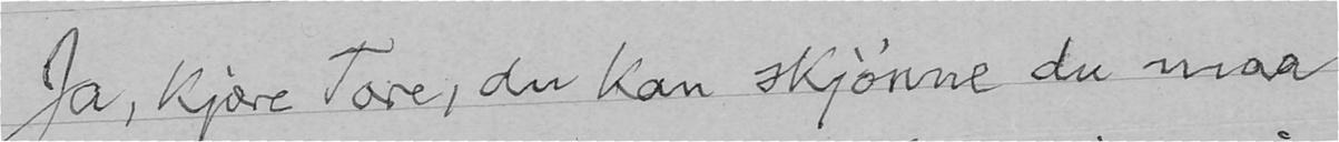Ja, kjære Tore, du kan skjønne du maa
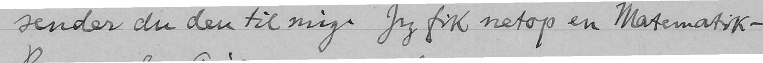sender du den til mig. Jeg fik netop en Matematik-
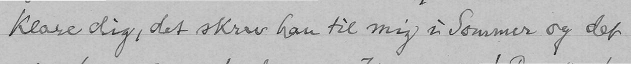klare dig, det skrev han til mig i Sommer og det
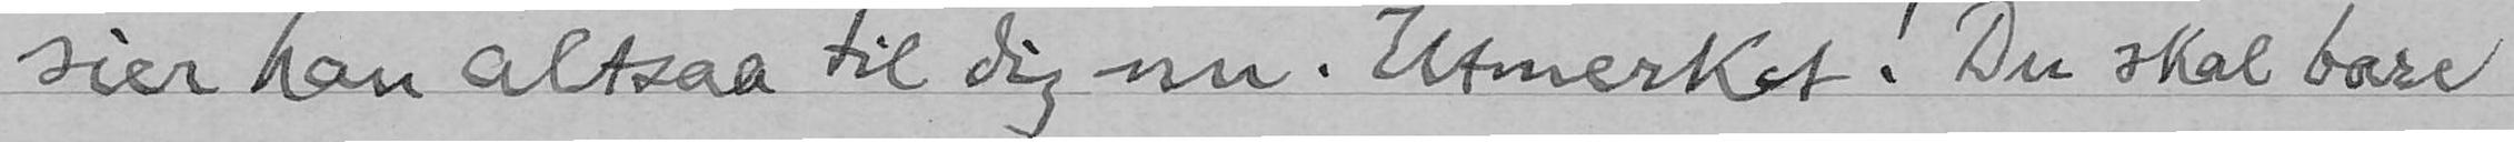sier han altsaa til dig nu. Utmerket! Du skal bare
